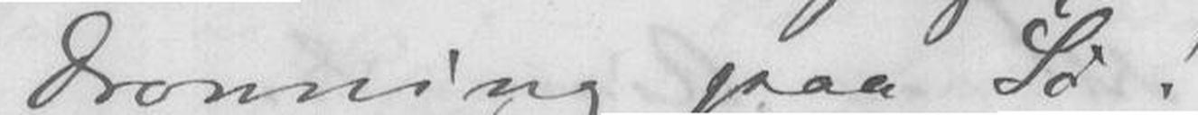Dronning paa Sø!
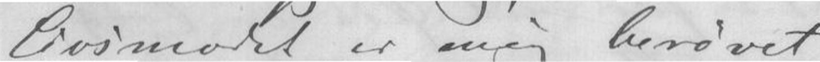Livsmodet er mig berøvet
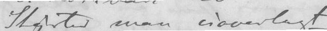Styrter man uoverlagt
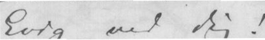Evig ved dig!
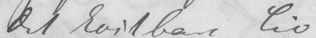det Evigbare Liv
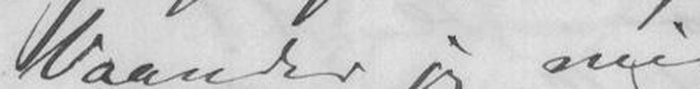Vaander jeg mig;
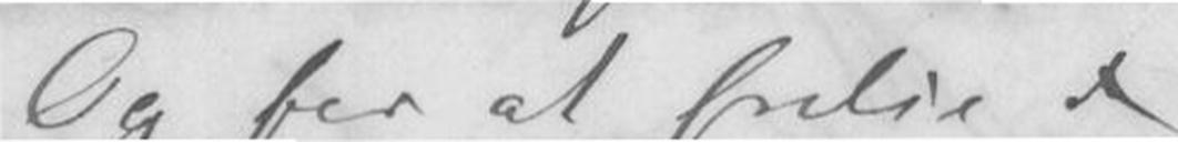Og for al frelse -
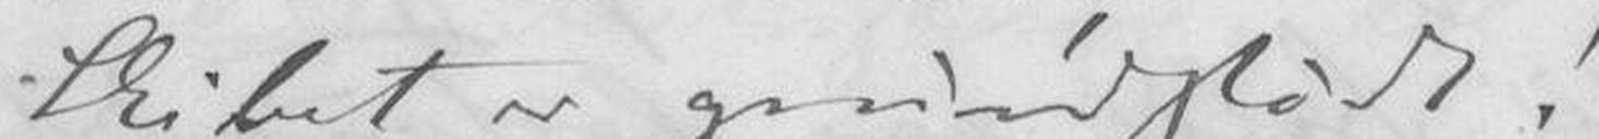Skibet er grundstødt! -
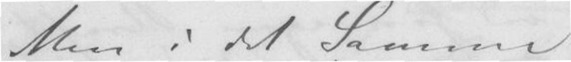Men i det Samme
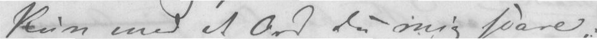Kun med et Ord du mig svare, -
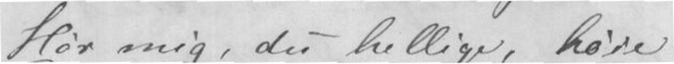Hør mig, du hellige, høie
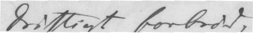dristigt forbrød,
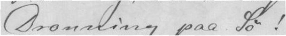Dronning paa Sø!
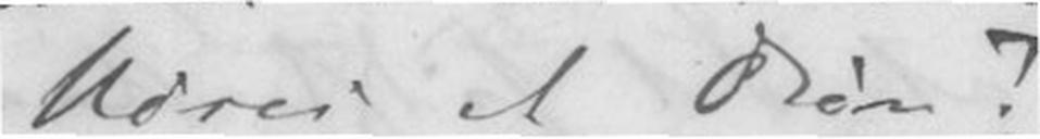høres et drøn!
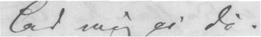Lad mig ei dø!
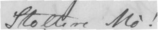Stoltere Mø!
